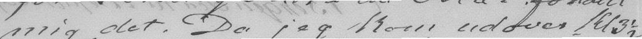mig det. Da jeg kom udover Kl.3½,
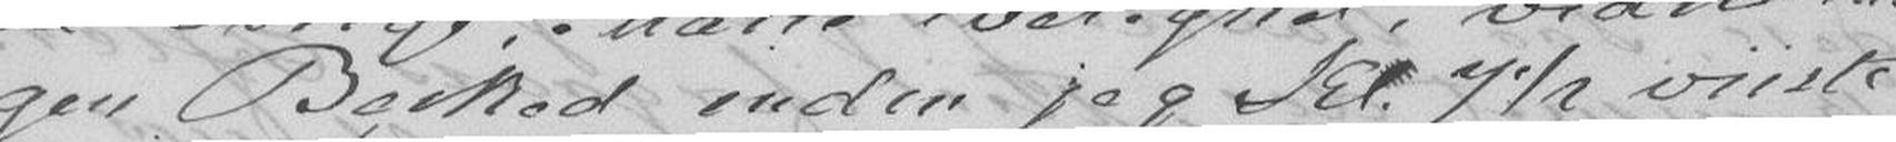gen Besked endnu jeg Kl 7½ viiste
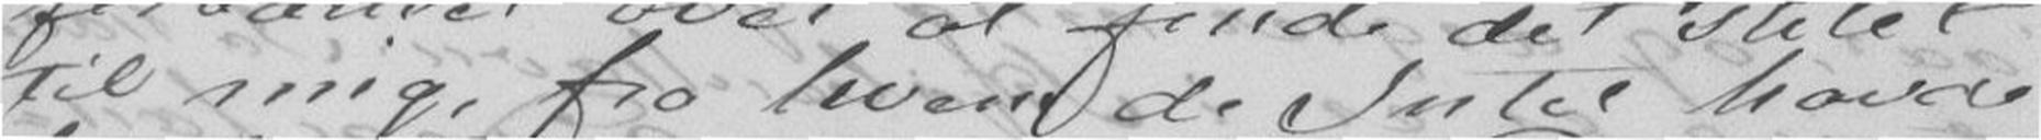til mig, fra hvem de Intet havde
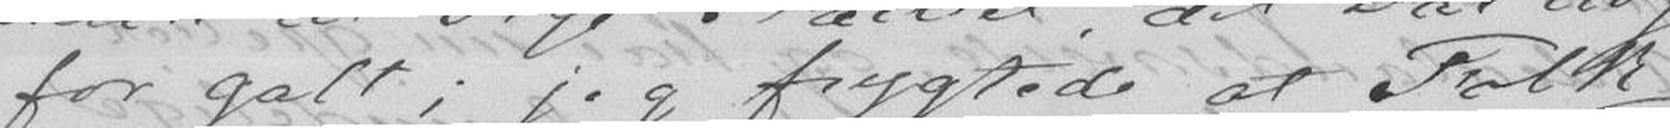for galt; jeg frygtede at Folk
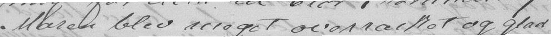Maren blev meget overrasket og glad
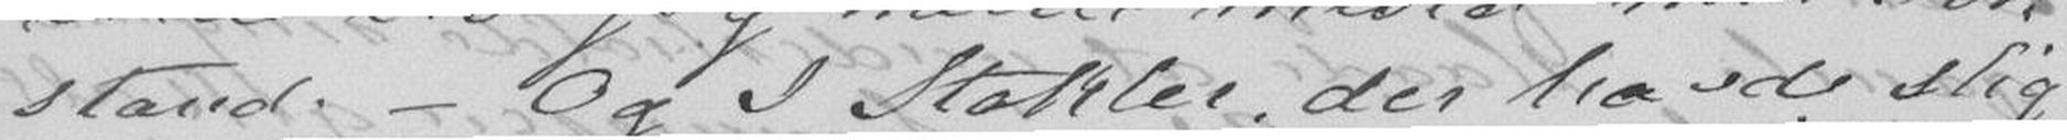stand. - Og I Stakler der havde slig
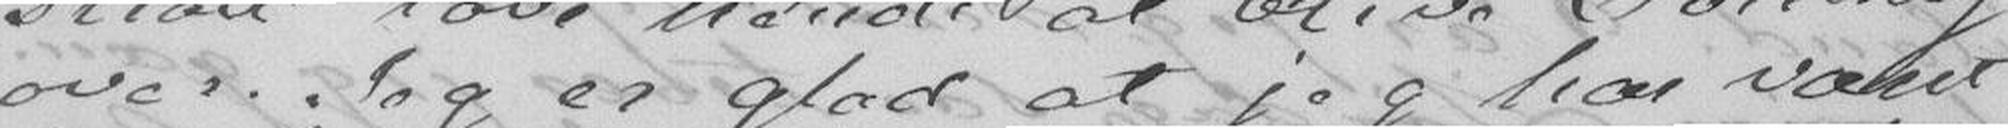over. Jeg er glad at jeg har været
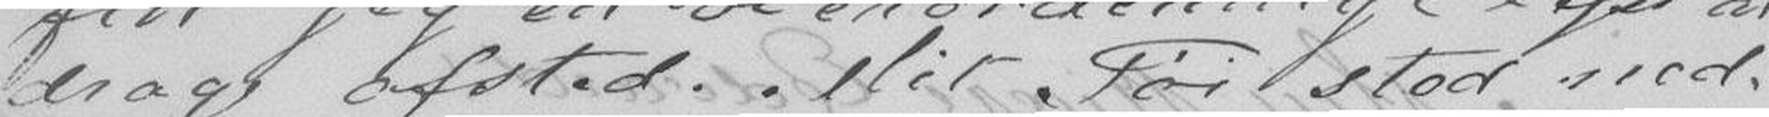drage afsted. Mit Tøi stod ind-
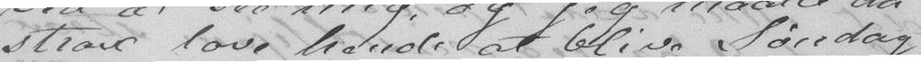strax love hende at blive Søndag
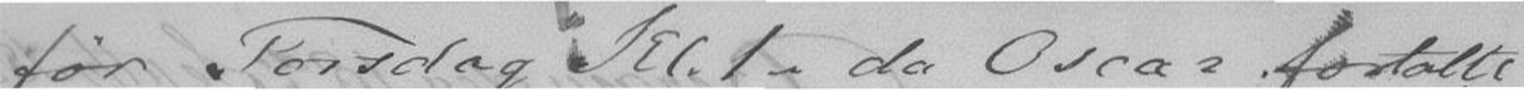før Torsdag Kl.1 - da Oscar fortalte
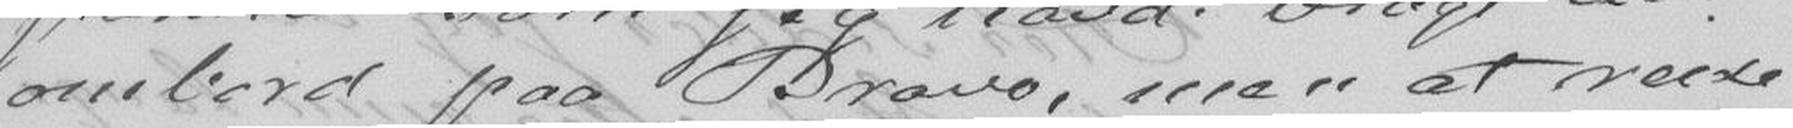ombord paa Bravo, men at reise
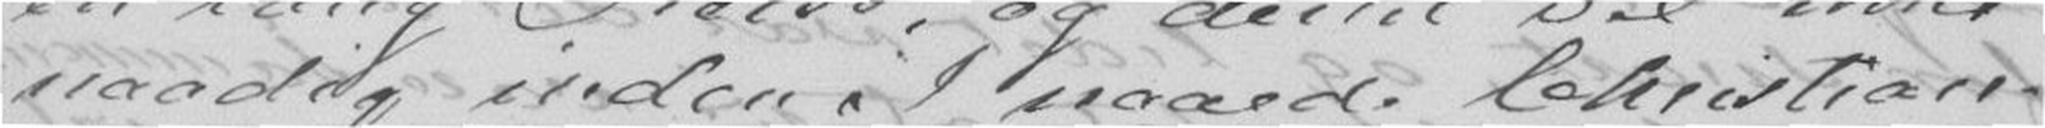naadig inden I naaede Christian-
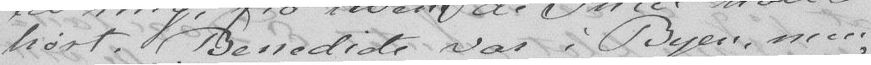hørt. Benedicte var i Byen, men
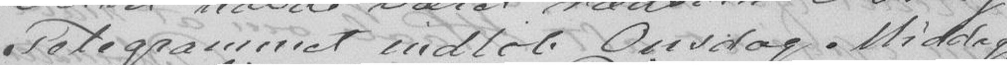Telegrammet indløb Onsdag Middag.
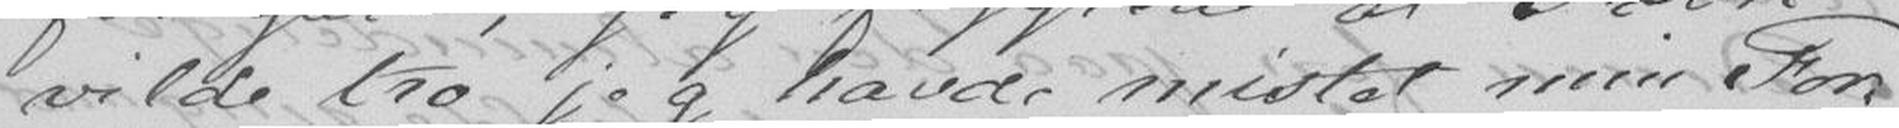vilde tro jeg havde mistet min For-
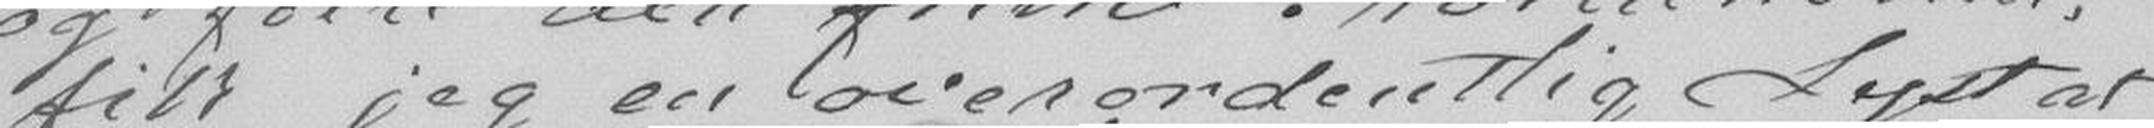fik jeg en overordentlig Lyst at
